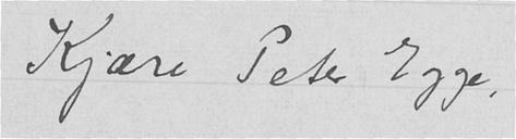Kjære Peter Egge,
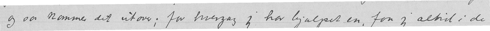og saa kommer det utover; for hvergang jeg har hjulpet en, faar jeg altid i de
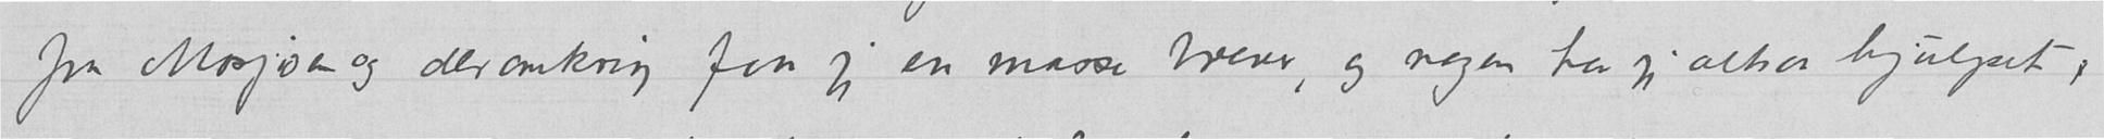fra Mosjøen og der omkring faar jeg en masse brever, og nogen har jeg altsaa hjulpet
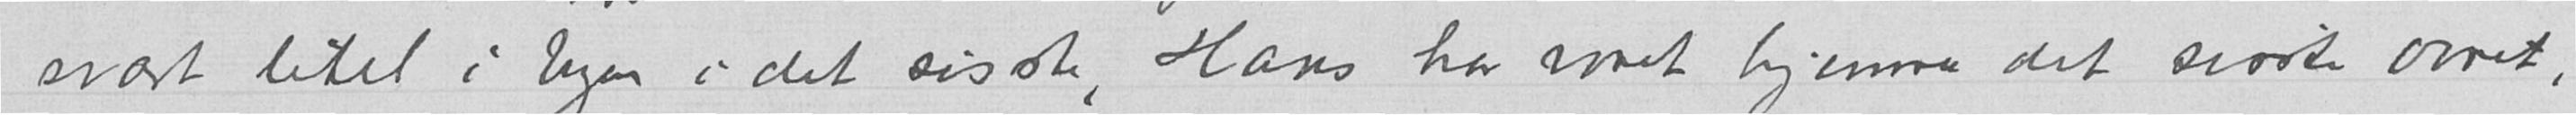svært litet i byen i det sisste, Hans har været hjemme det sidste aaret,
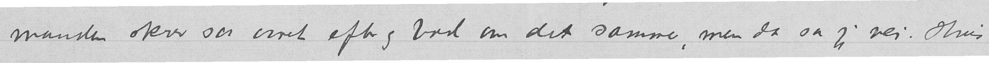manden skrev saa aaret efter og bad om det samme, men da sa jeg nei. Hvis
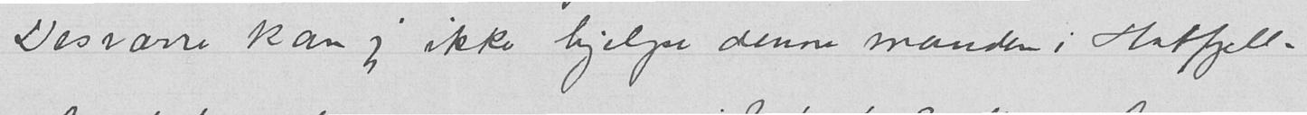Desværre kan jeg ikke hjelpe denne manden i Hatfjell-
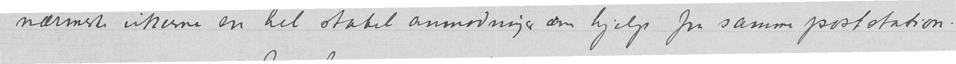nærmeste ukerne en hel stabel anmodninger om hjelp fra samme poststation.
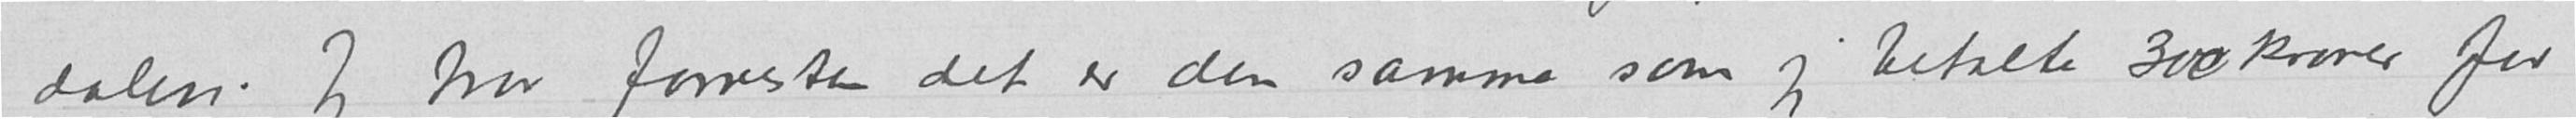dalen. Jeg tror forresten det er den samme som jeg betalte 300 kroner for
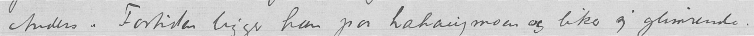Anders. Fortiden ligger han paa Lahaugmoen og liker sig glimrende.
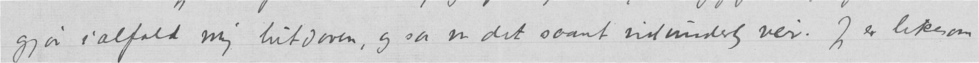gjør ialfald mig lutdoven, og saa var det saant vidunderlig veir. Jeg er likesom
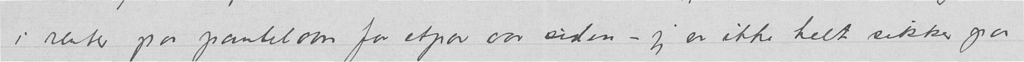i renter paa pantelaan for etpar aar siden – jeg er ikke helt sikker paa
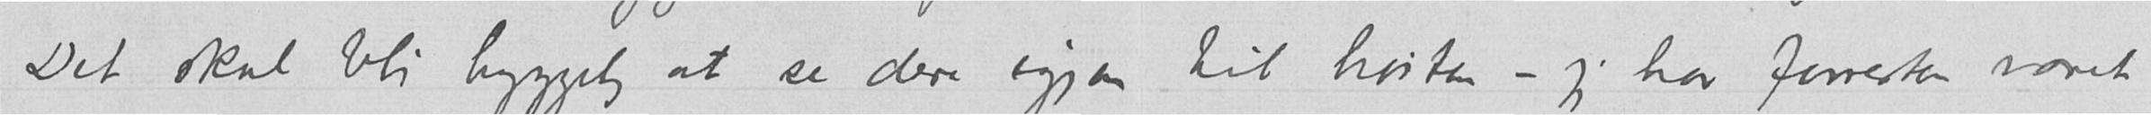Det skal bli hyggelig at se dere igjen til høsten – jeg har forresten været
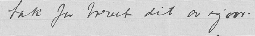tak for brevet dit av igaar.
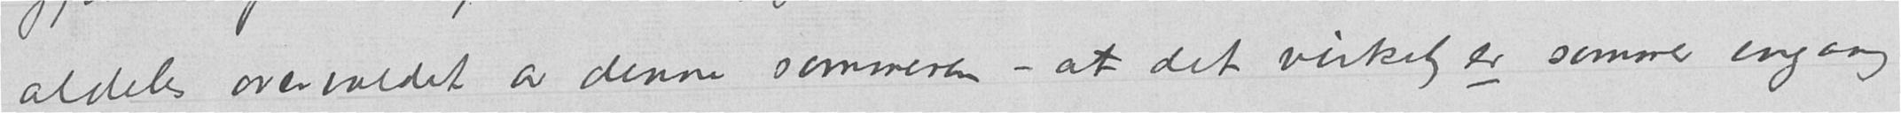aldeles overvældet av denne sommeren – at det virkelig er sommer engang
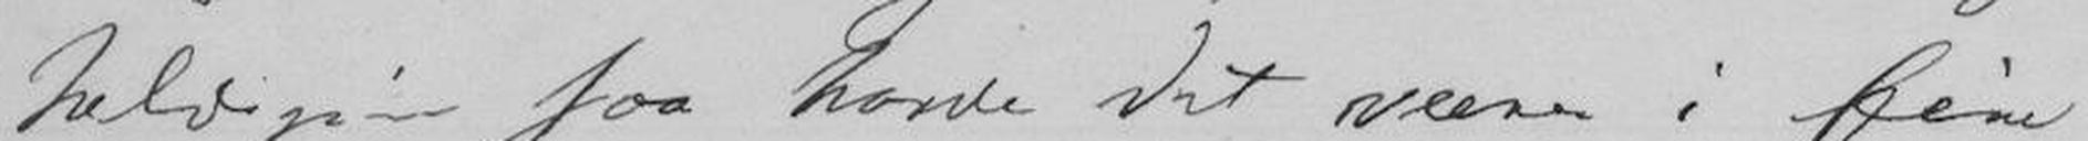held jo - saa havde det være i sine
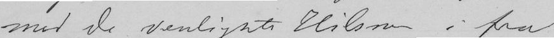med de venlighte Hilsen i fra
